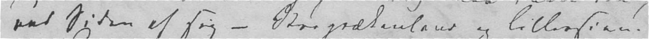ved Siden af sig - Storgrækenland og Lilleasien.
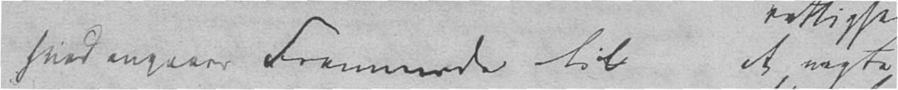hvad angaaer Fremmede til at nægte
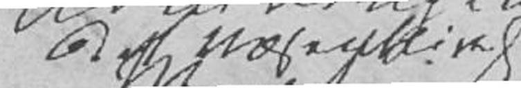det Væsentlige
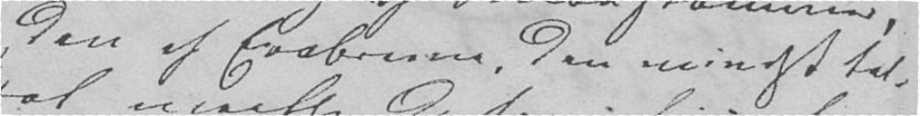den af Erobrerne, den mindst tal-
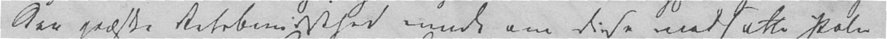den græske Retsbevidsthed kunde om disse modsatte Poler
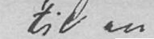til en
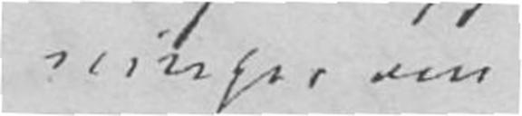ninger om
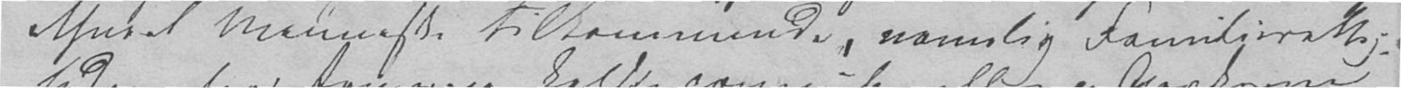ethvert Menneske tilkommende, nemlig Familierettig-
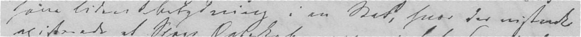have liden Betydning i en Stat, hvor der vistnok
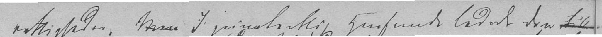rettigheder. Men I privatretlig Henseende ledede den til
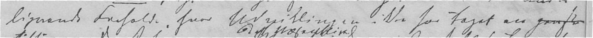lignende Forholde, hvor Udviklingen ikke har taget en ganske
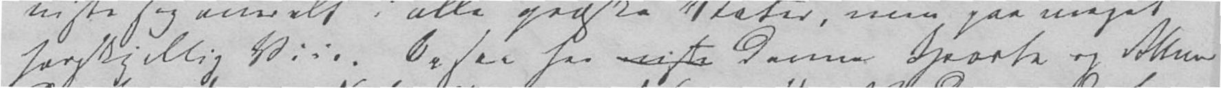forskjellig Viis. Ogsaa her viste danner Sparta og Athen
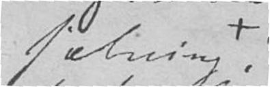sætning
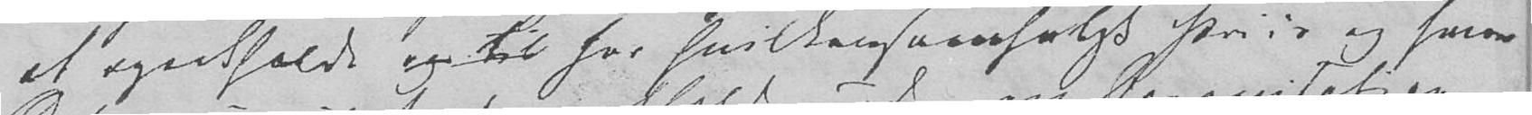at egenholde og til for hvilkensomhelst Priis og hvem
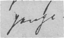penge.
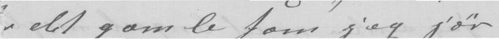det gamle som jeg jør
